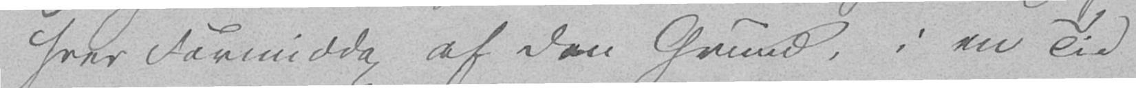hver Formiddag af den Grund, i en Tid
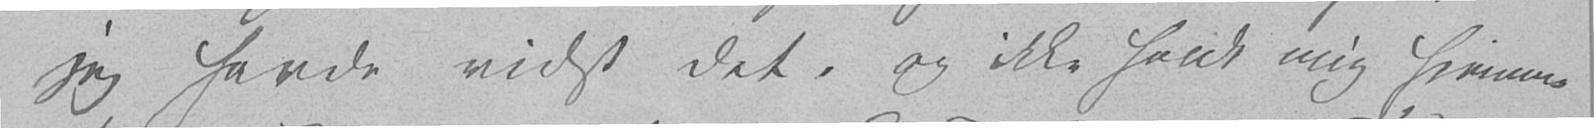jeg havde vidst det, og ikke holdt mig hjemme
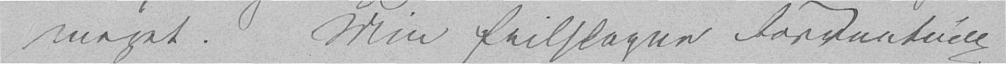meget. Min feilslagne Forventning
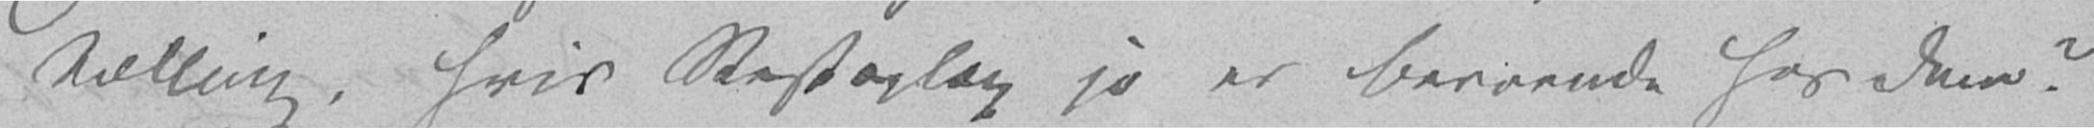tælling, hvis Restoplag jo er beroende hos Dem?
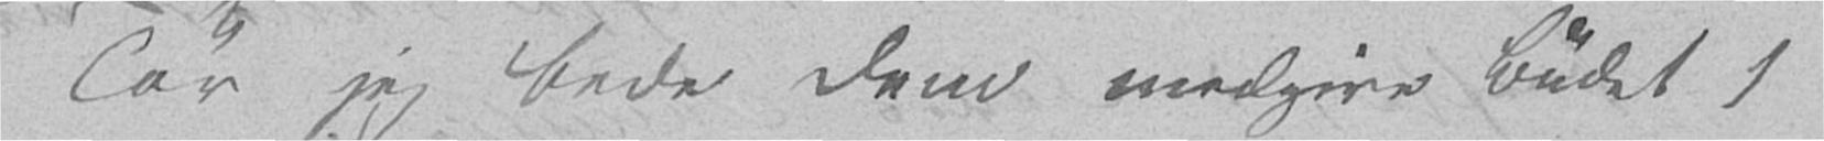Tør jeg bede Dem medgive Budet 1
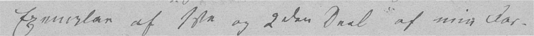Exemplar af 1ste og 2den Deel af min For
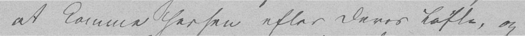at komme herhen efter Deres Løfte, og
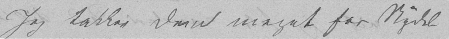Jeg takker Dem meget for Nydel
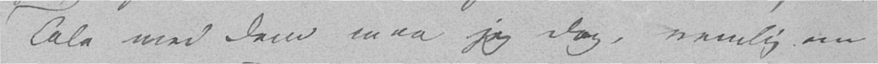Tale med Dem maa jeg dog, nemlig om
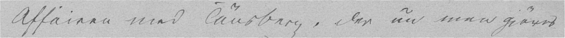Affairen med Tønsberg, der nu maa gjøres
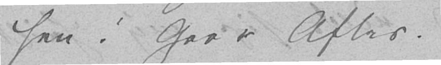sen i Gaar Aftes.
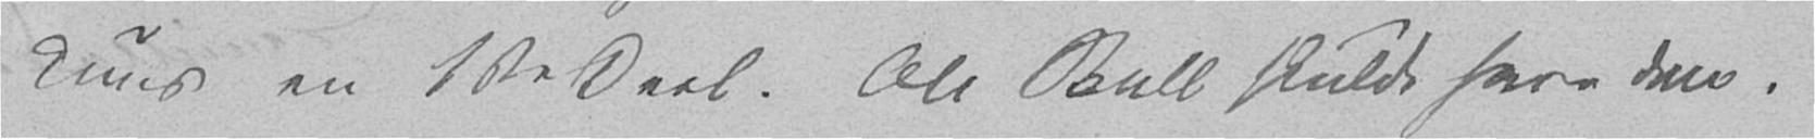kuns en 1ste Deel. Ole Bull skulde have den.
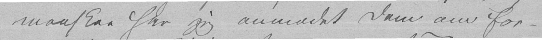maaskee har jeg anmodet Dem om for-
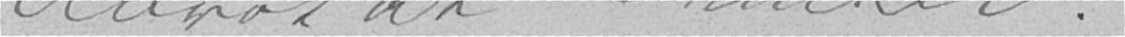Advokat Dunker.
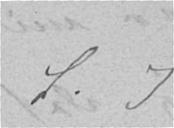S. T
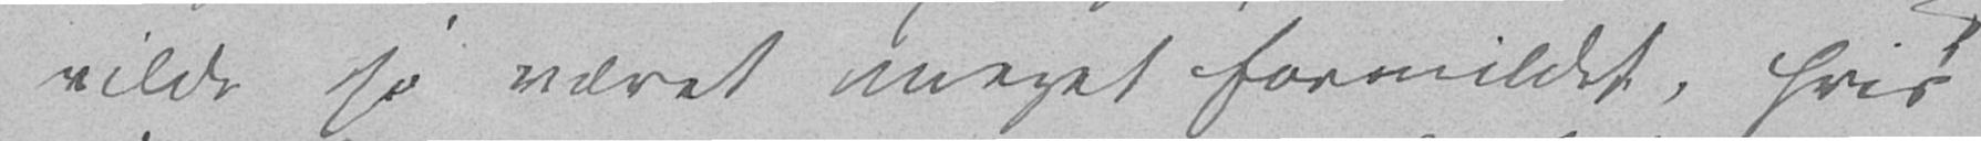vilde jo været meget formildet, hvis
